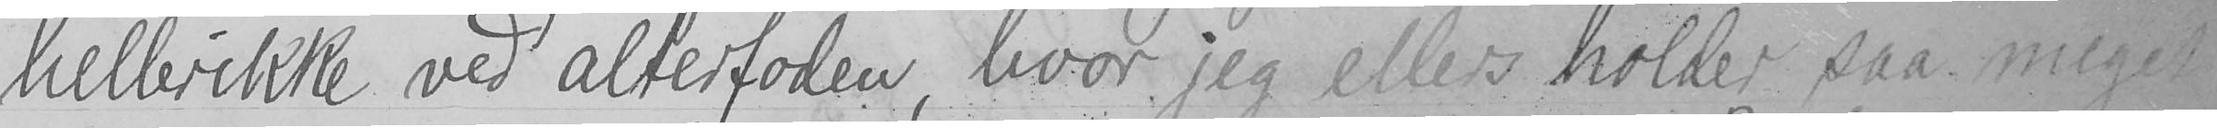hellerikke ved alterfoden, hvor jeg ellers holder saa meget
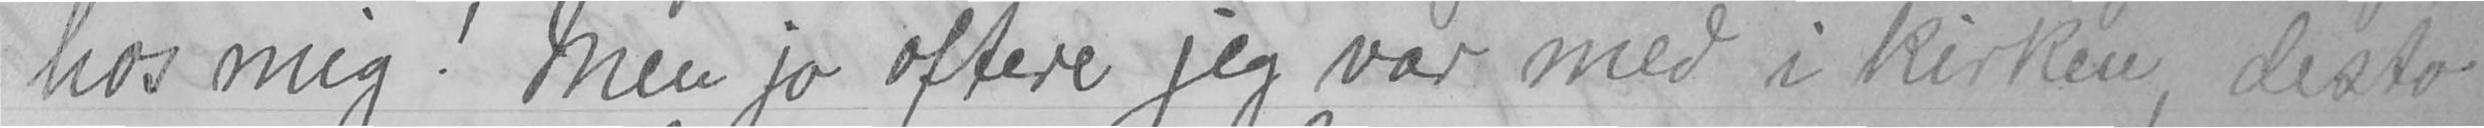hos mig! Men jo oftere jeg var med i kirken, desto
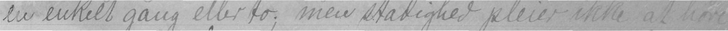en enkelt gang eller to; men statighed pleier ikke at høre
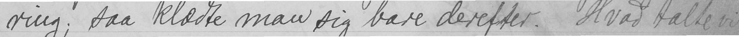ring; saa klædte man sig bare derefter. Hvad talte vi
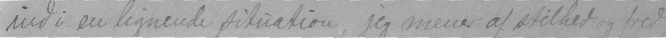ind i en lignende situation, jeg mener af stilhed og fred,
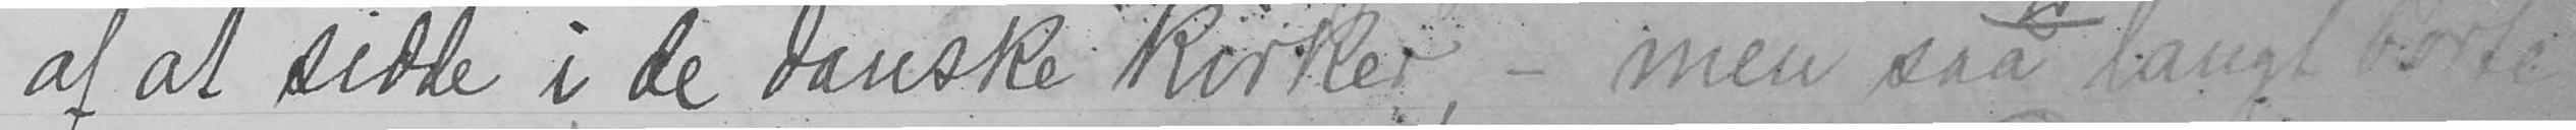af at sidde i de danske kirker, - men saa langt borte
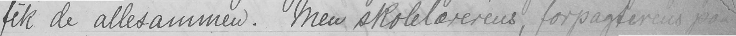fik de allesammen. Men skolelærerens, forpagterens paa
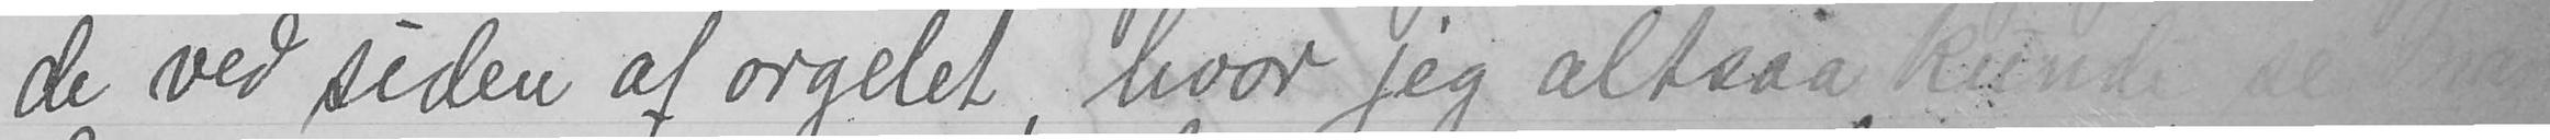de ved siden af orgelet, hvor jeg altsaa kunde se Marie
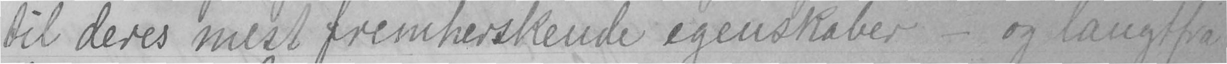til deres mest fremherskende egenskaber - og langt fra
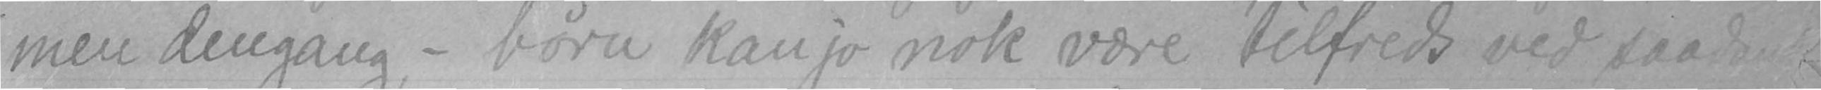men dengang, - børn kan jo nok være tilfreds ved saadant
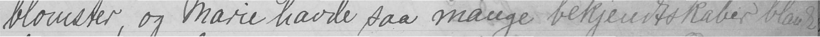blomster, og Marie havde saa mange bekjendtskaber blandt
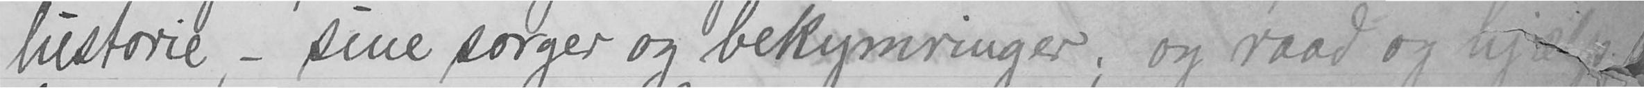historie, - sine sorger og bekymringer; og raad og hjælp
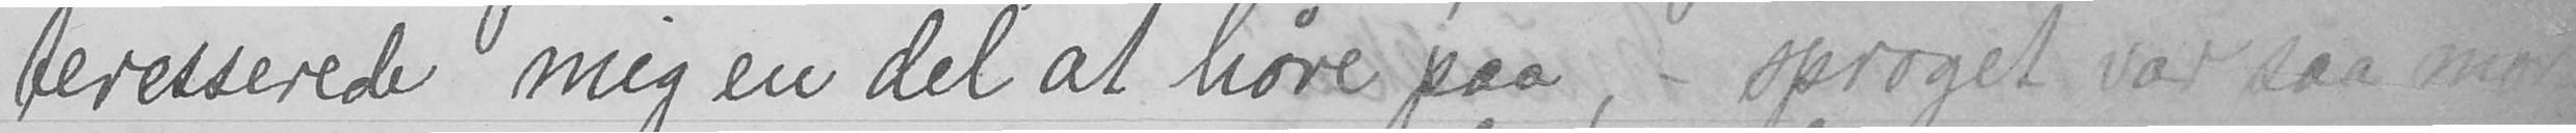teresserede mig en del at høre paa, - sproget var saa mor-
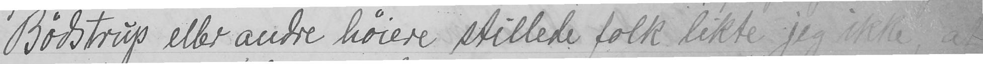Bødstrup eller andre høiere stillede folk likte jeg ikke, at
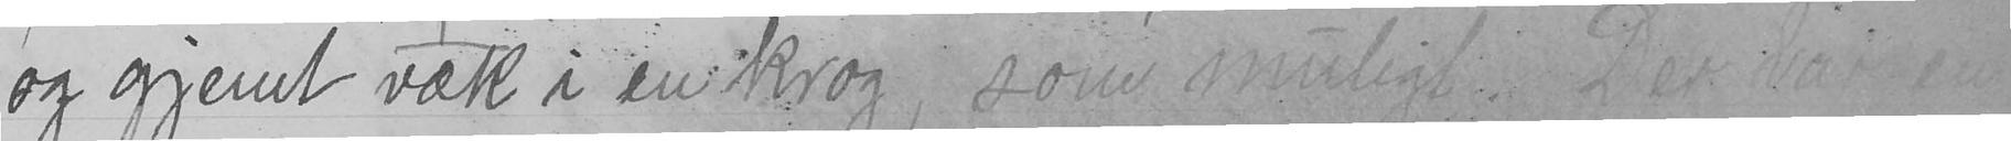og gjemt væk i en krog, som muligt. Der var en af
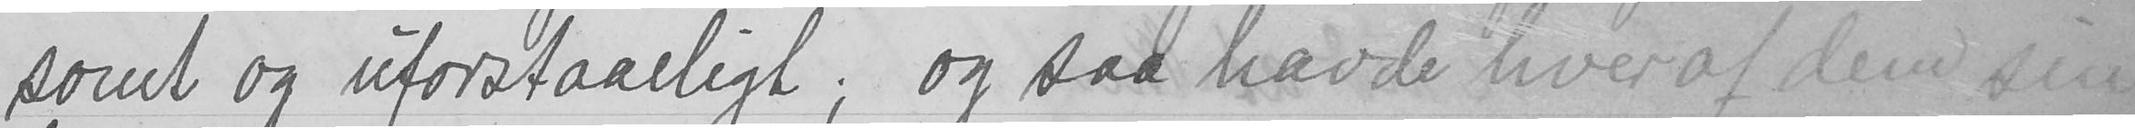somt og uforstaaeligt; og saa havde hver af dem sin
